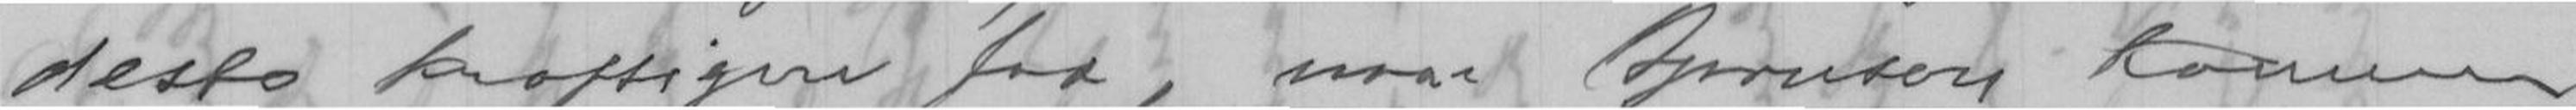desto kraftigere fra, naar Bjørnson konner
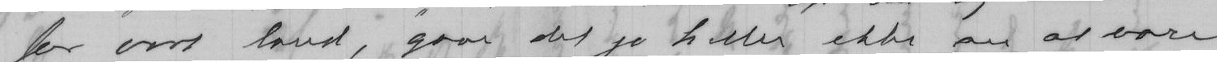for vort land, gaar det jo heller ikke an at være
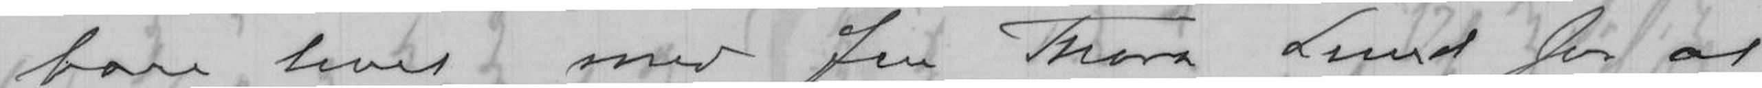bare livet med fru Thora Lund for at
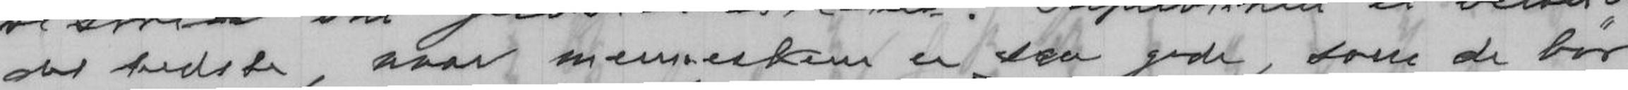det bedste, naar menneskene er saa gode, som de bør
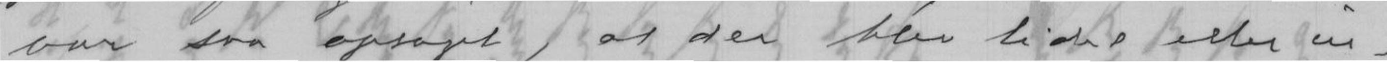var saa optaget, at der blev lidet eller in-
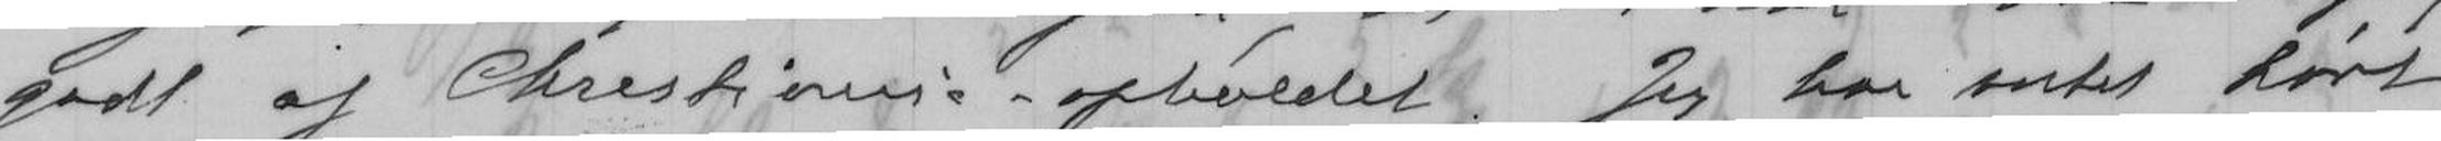godt af Christiania-opholdet. Jeg har intet hørt
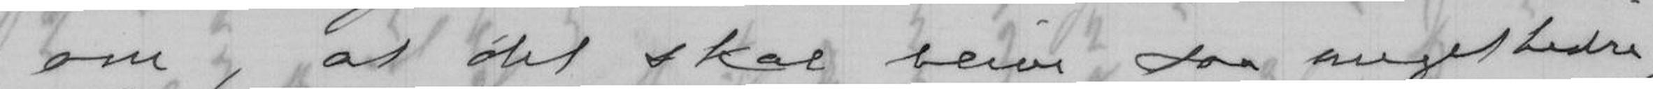om, at det skal blive saa meget bedre
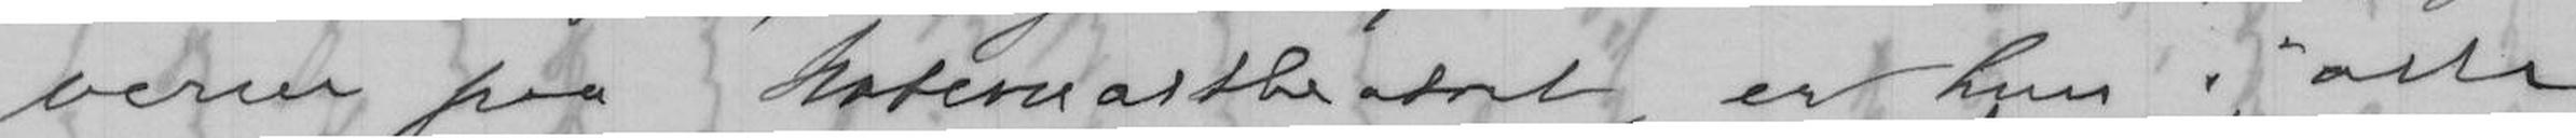verne paa Nationaltheatret, er hun i alle
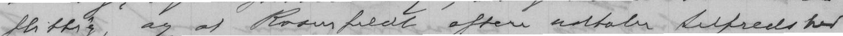flittig, og at Rosenfeldt oftere udtaler tilfredshed
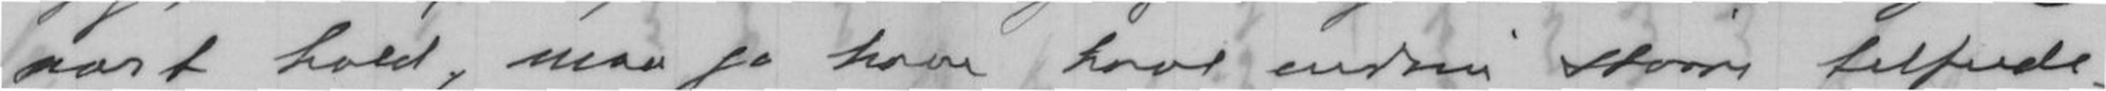nært hold, maa jo have havt endnu større tilfreds-
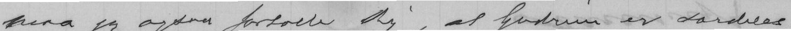maa jeg ogsaa fortalle Dig, at Gudrun er særdeles
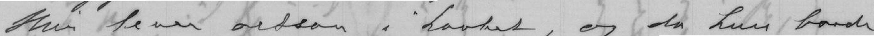Hun lever altsaa i haabet, og da hun baade
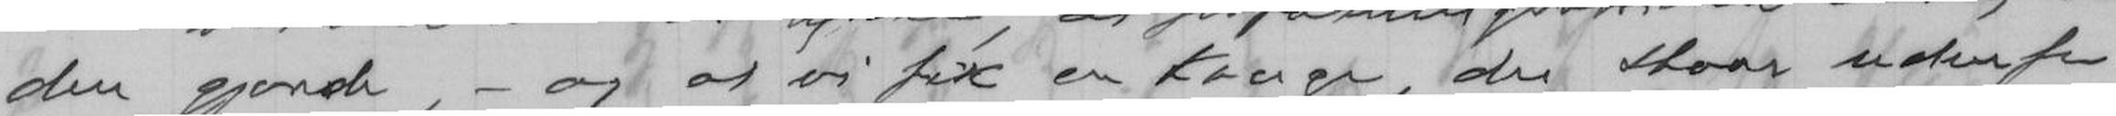den gjorde, - og at vi fik en konge, der staar udenfor
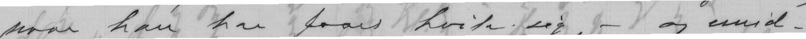naar han har faaet hvile sig, - og imid-
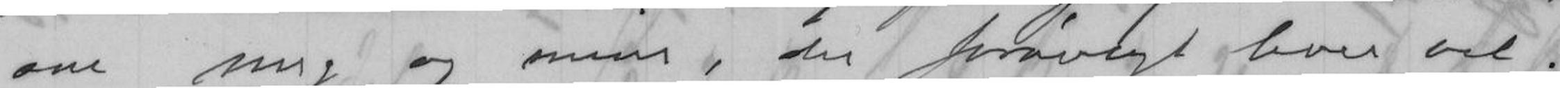om mig og mine, der forøvrigt lever vel.
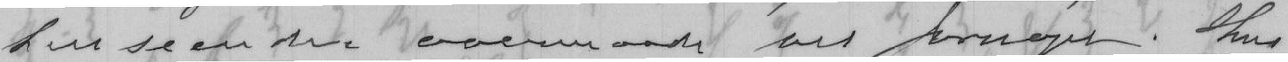henseende overmaade ved poenget. Hun
